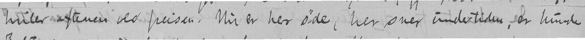hviler aftenen ved peisen. Nu er her øde, her sner undertiden, er hunde-
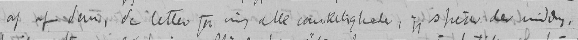af af dem, de letter for mig alle vanskeligheder, jeg spiser der middag,
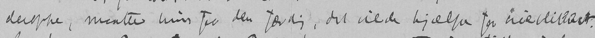deroppe, maatte hun faa den færdig, det vilde hjælpe for øieblikket.
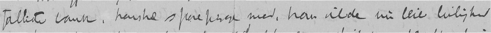fallite bank, kanske sparepænge med, han vilde nu leie leilighed
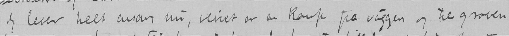Jeg lever helt ensom nu, veiret er en kamp fra vuggen og til graven
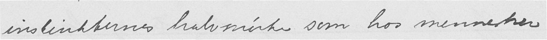instinkternes halvmørke som hos mennesker
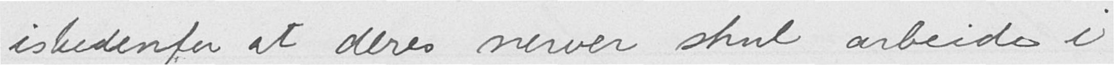istedenfor at deres nerver skal arbeide i
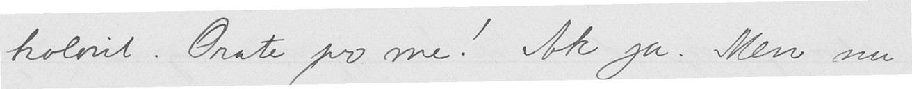kolorit. Orate pro me! Ak ja. Men nu
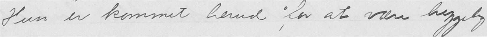Hun er kommet herud "for at være hyggelig
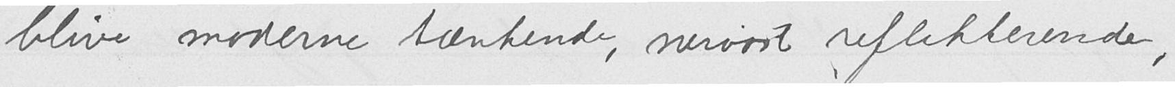blive moderne tænkende, nervøst reflekterende,
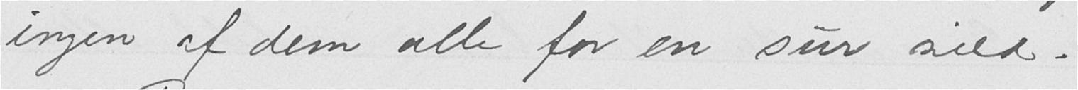ingen af dem alle for en sur sild.
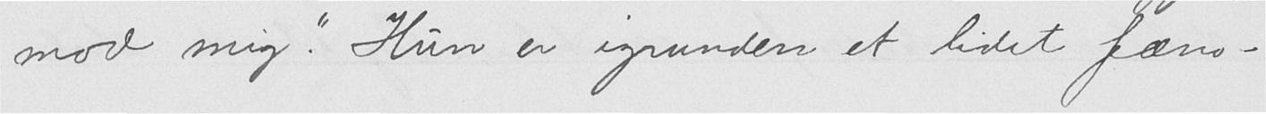mod mig". Hun er igrunden et lidet fæno-
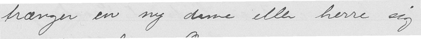trænger en ny dame eller herre sig
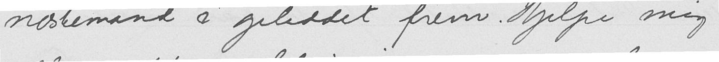næstemand i geleddet frem. Hjelpe mig
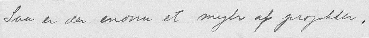Saa er der endnu et mylr af projekter,
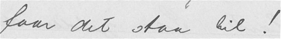faar det staa til!
